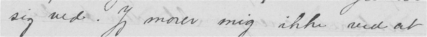sig ved. Jeg morer mig ikke ved at
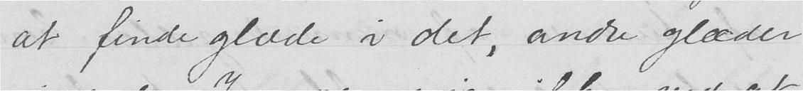at finde glæde i det, andre glæder
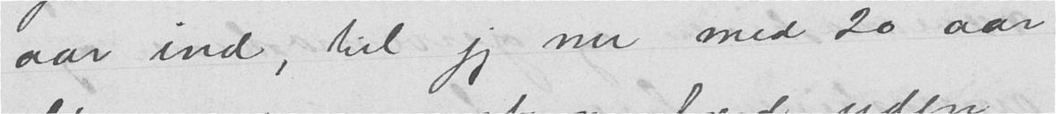aar ind, til jeg nu med 20 aar
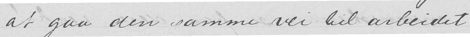at gaa den samme vei til arbeidet
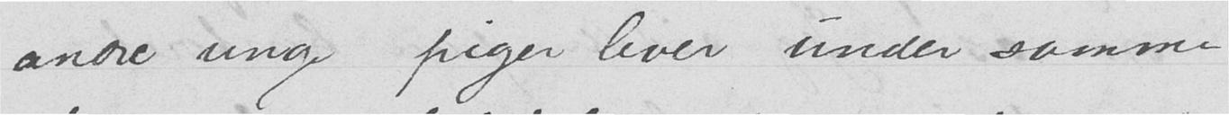andre unge piger lever under samme
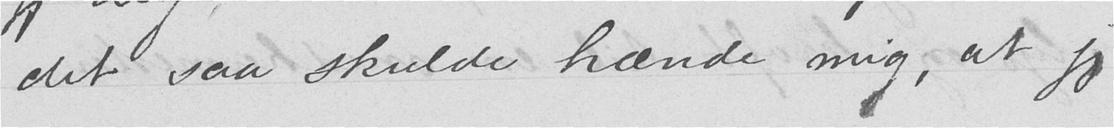det saa skulde hænde mig, at jeg
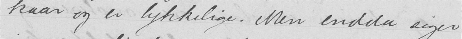kaar og er lykkelige. Men endda siger
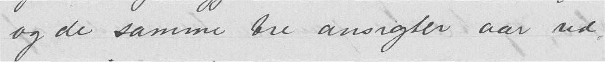og de samme tre ansigter aar ud,
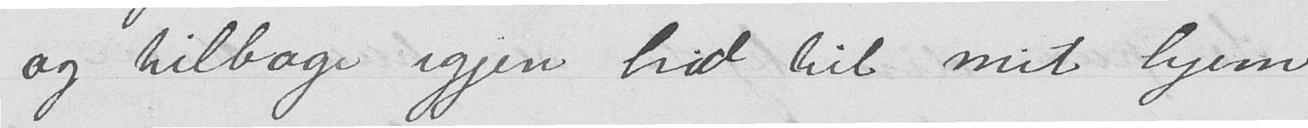og tilbage igjen hid til mit hjem
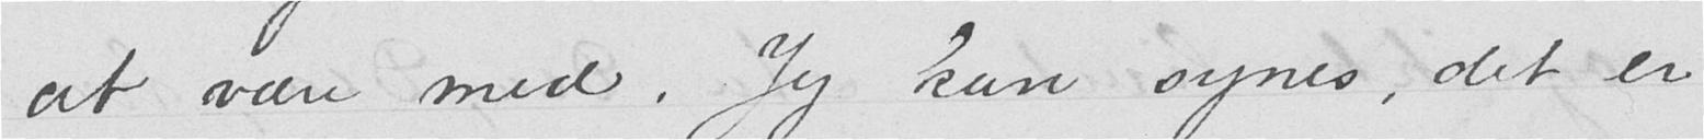at være med. Jeg kan synes, det er
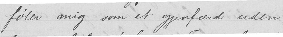føler mig som et gjenfærd uden
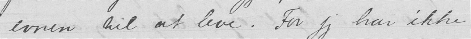evnen til at leve. For jeg har ikke
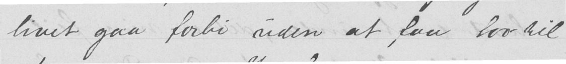livet gaa forbi uden at faa lov til
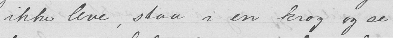ikke leve, staa i en krog og se
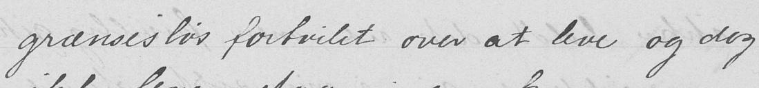grænsesløs fortvilet over at leve og dog
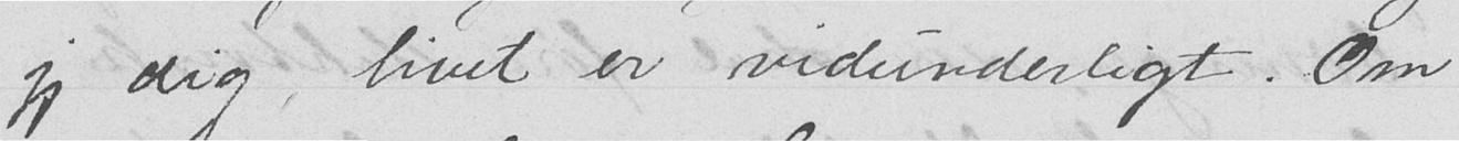jeg dig, livet er vidunderligt. Om
